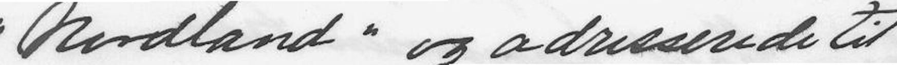"Nordland" og adresserede til
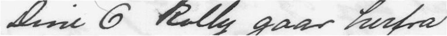Dine 6 kolly gaar herfra
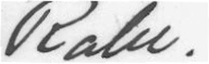Rabe. -
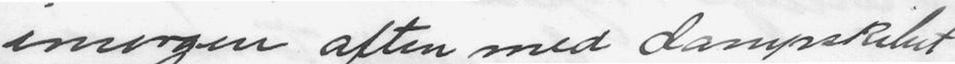imorgen aften med dampskibet
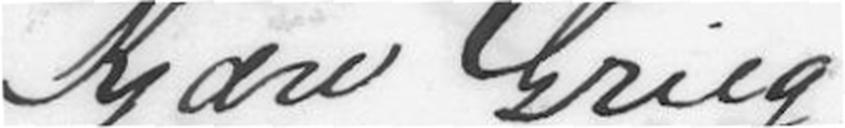Kjære Grieg
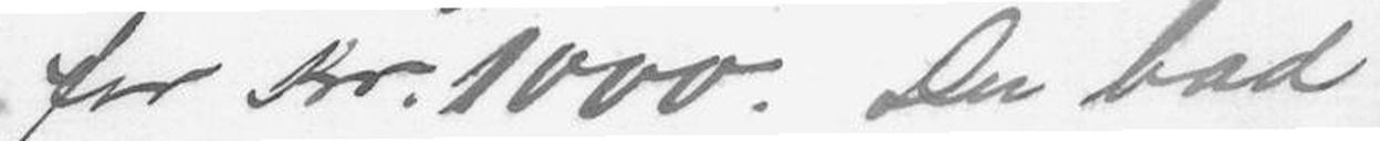for Kr. 1000. Du bad
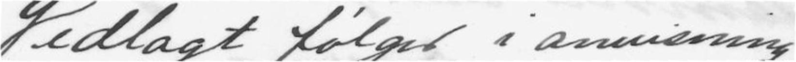Vedlagt følger i anvisning
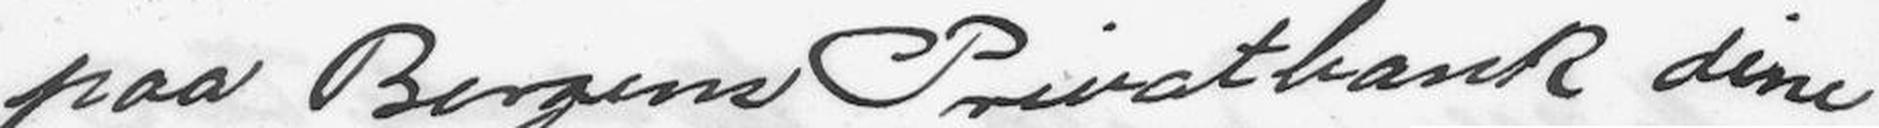paa Bergens Privatbank dine
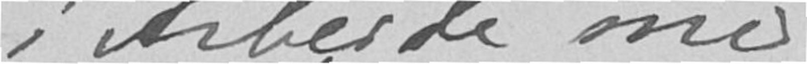i Arbeide med
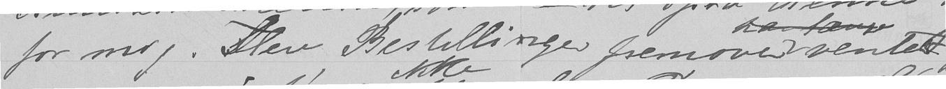for mig. Mine Bestillinger fremover venter
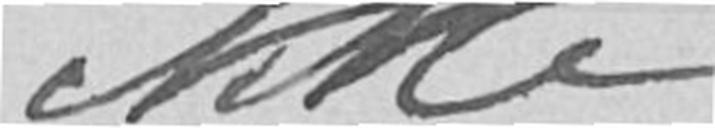ikke
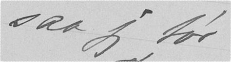saa jeg tør
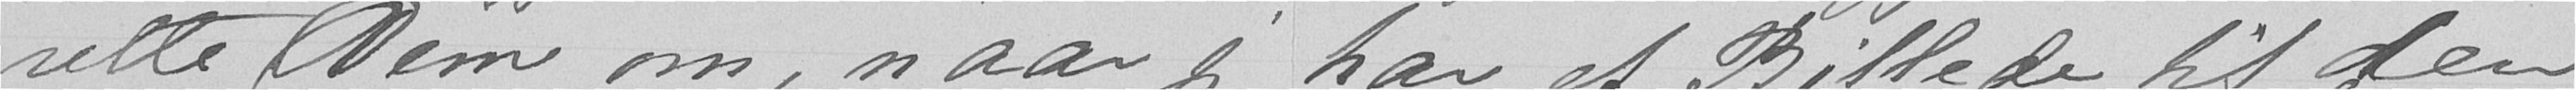rette Dem om, naar jeg har et Billede til den
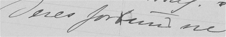Deres forbundne
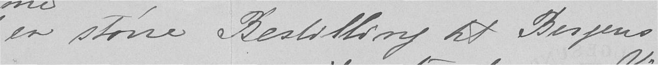en større Bestilling til Bergens
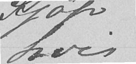hvis
